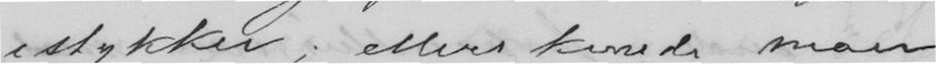istykker; ellers kunde man
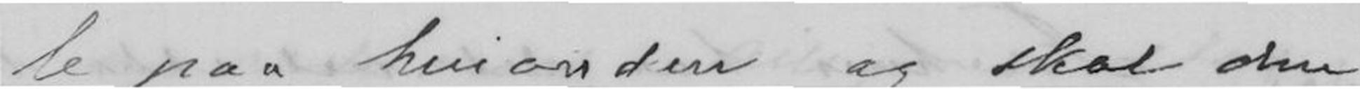le paa hinanden og skal den
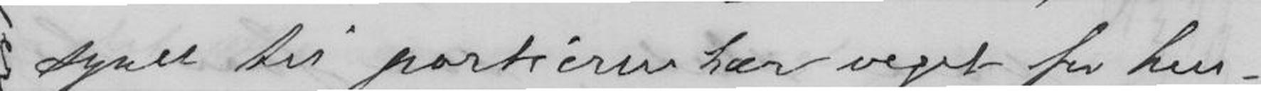synet til partiene har veget for hen-
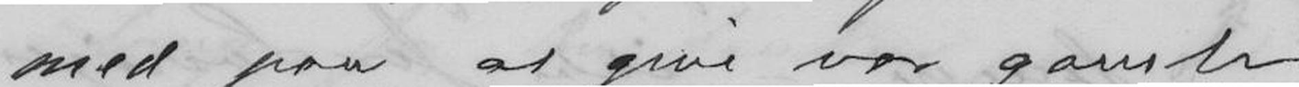med paa et at give vor gamle
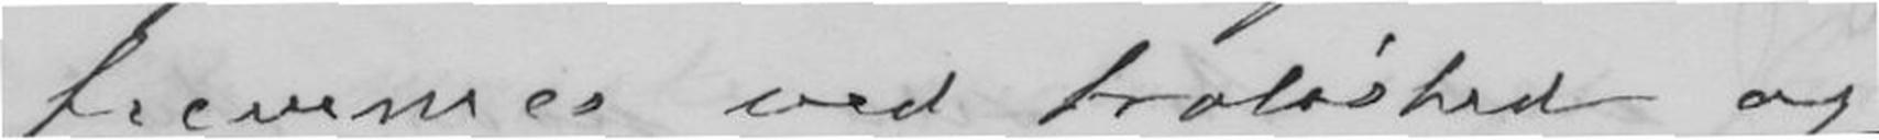fremmes ved troløshed og
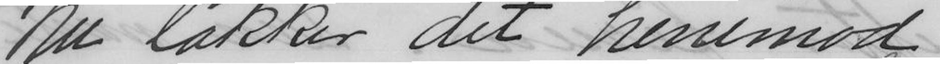Nu lakker det henimod
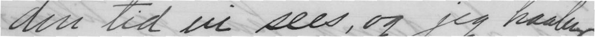den tid vi sees, og jeg haaber
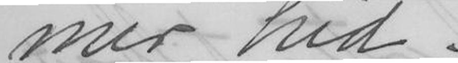mer hid.
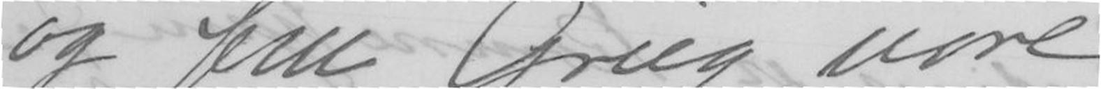og fru Grieg vore
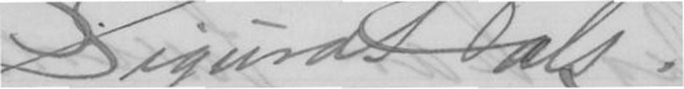Sigurd Hals
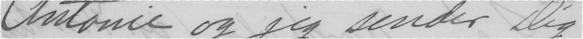Antonie og jeg sender Dig
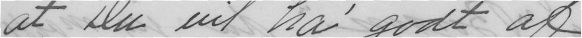at Du vil ha' godt af
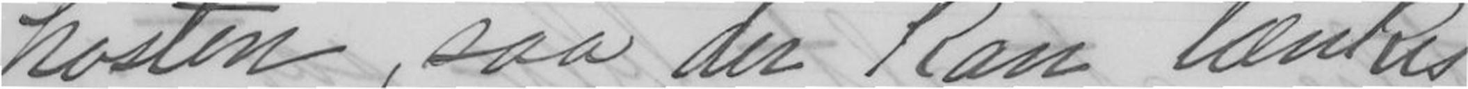høsten, saa der kan tænkes
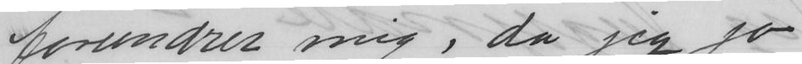forundrer mig, da jeg jo
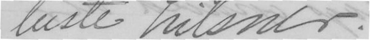beste hilsner.
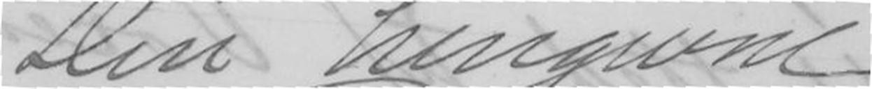Din hengivne
